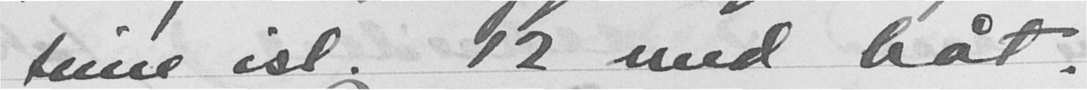time ist. 12 med båt.
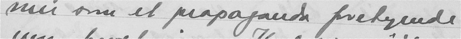mer som et propagandaforetagende
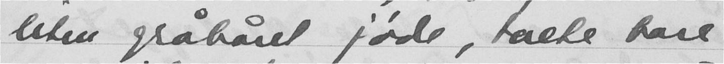liten grahåret jøde, talte bare
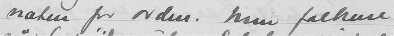natur for orden. Men folkene
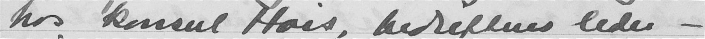hos konsul Hais, bedriftens leder -
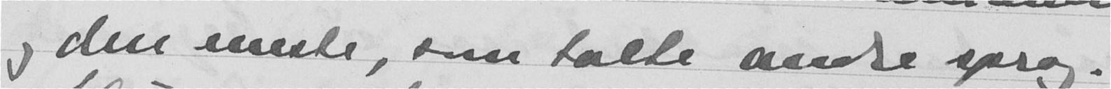og den eneste, som talte andre sprog.
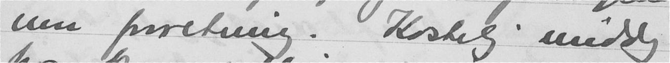enn forretning. Kostelig middag
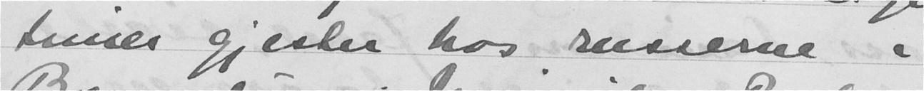timer gjester hos russerne i
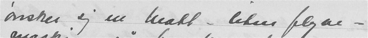ønsker sig en Moth - liten flyge-
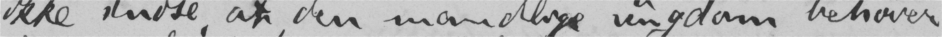ikke indse at den mandlige ungdom behøver
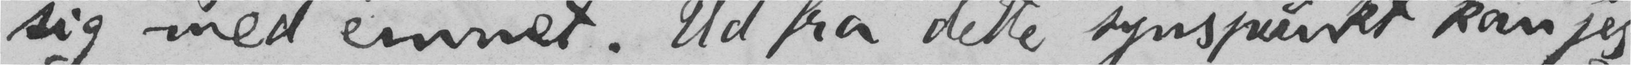sig med emnet. Ud fra dette synspunkt kan jeg
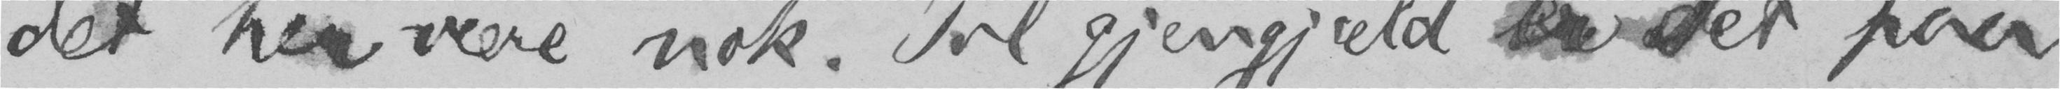det her være nok. Til gjengjæld er det paa
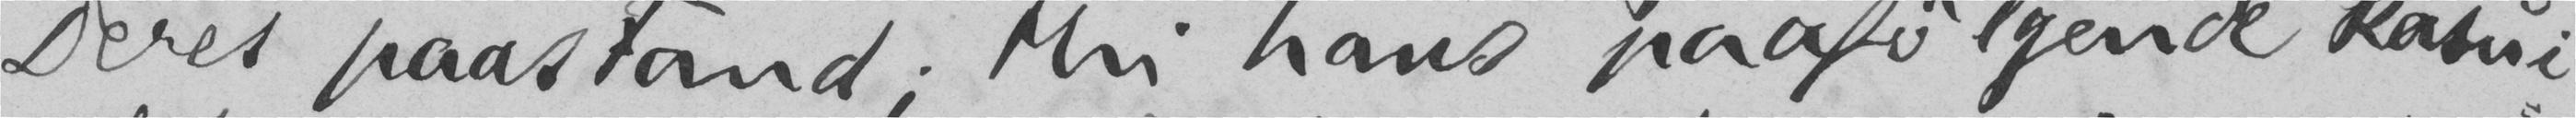Deres paastand; thi hans paafølgende kasui-
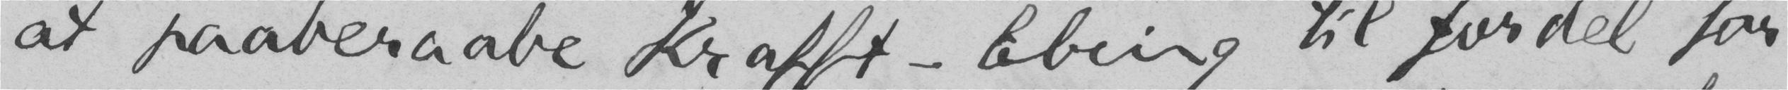at paaberaabe Krafft-Ebing til fordel for
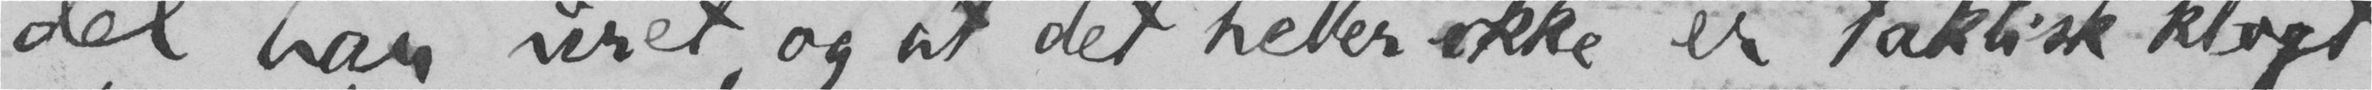del har uret, og at det heller ikke er taktisk klogt
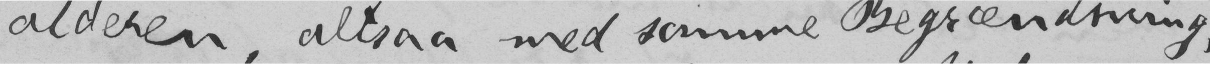alderen, altsaa med samme Begrændsning,
